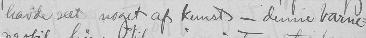havde seet noget af kunst - denne barne-
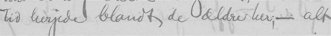tid herjede blandt de ældre her; - alt
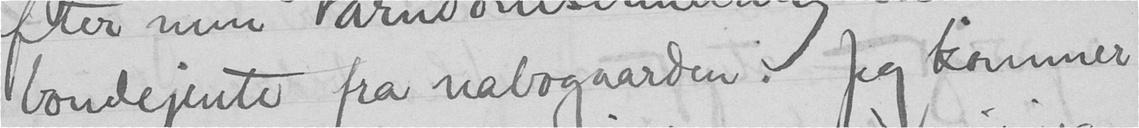bondejente fra nabogaarden. Jeg kommer
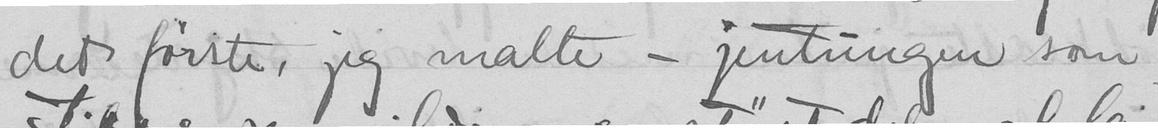det første, jeg malte - jentungen som
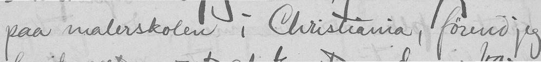paa malerskolen i Christiania - førend jeg
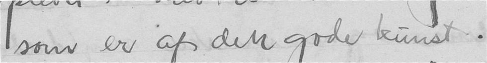som er af det gode kunst.
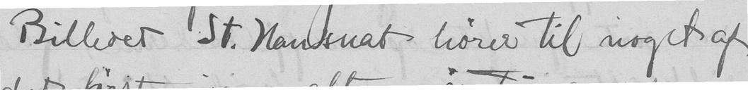Billedet St. Hansnat hører til noget af
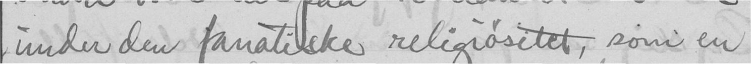under den fanatiske religiøsitet, som en
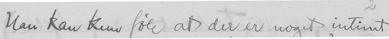Han kan kun føle at der er noget intimt
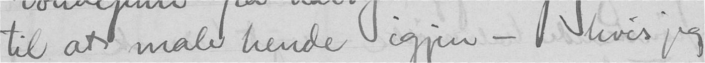til at male hende igjen, - hvis jeg
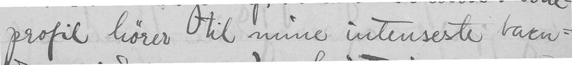profil hører til mine intenseste barn-
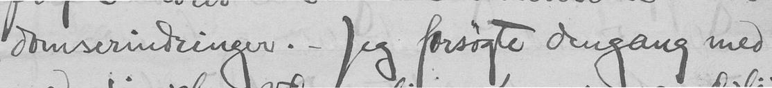domserindringer. - Jeg forsøgte dengang med
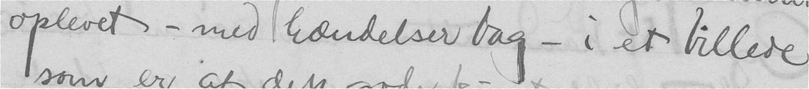oplevet - med hændelser bag - i et billede
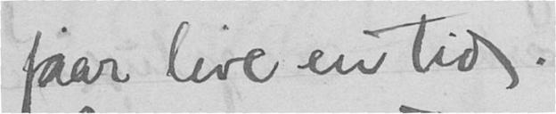faar live en tid.
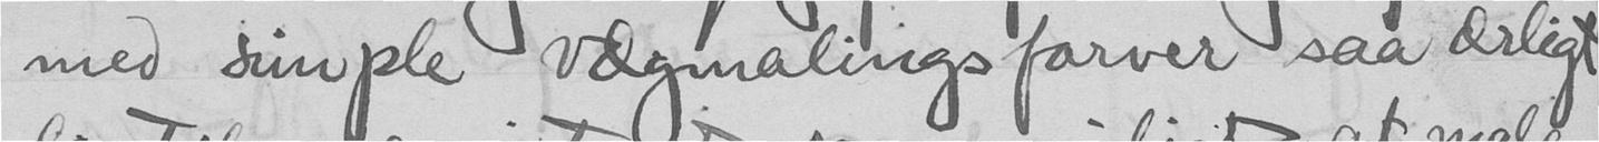med simple vægmalingsfarver saa ærligt
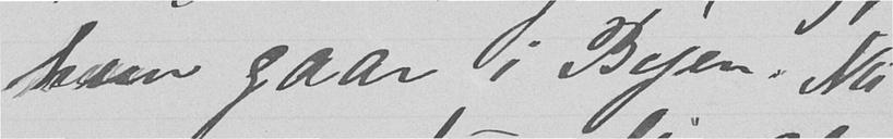han gaar i Byen. Nu
